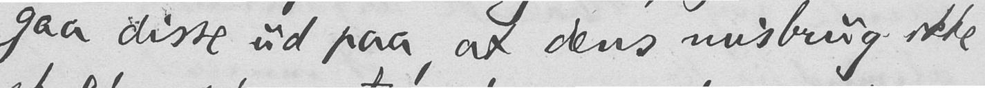gaa disse ud paa, at dens misbrug ikke
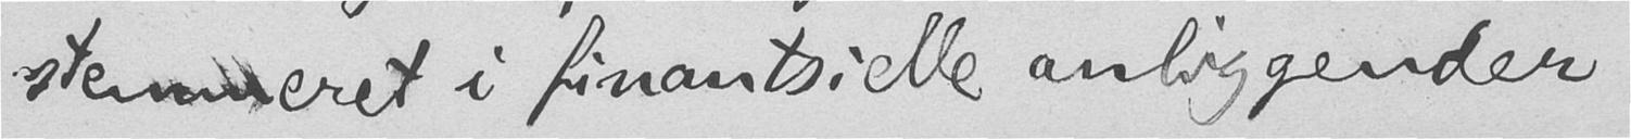stemmeret i finantsielle anliggender
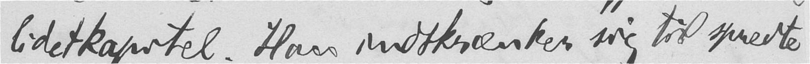lidet kapitel. Han indskrænker sig til spredte
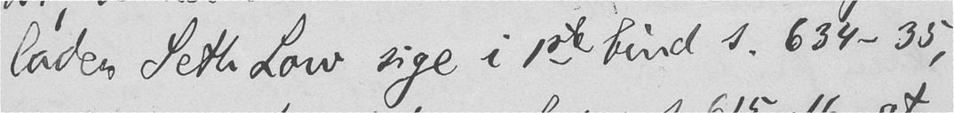lader Seth Low sige i 1ste bind s. 634-35,
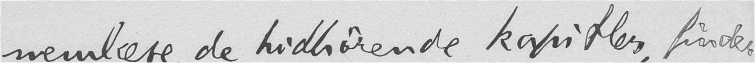nemlæse de hidhørende kapitler, finder
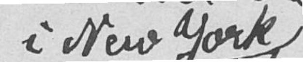i New York
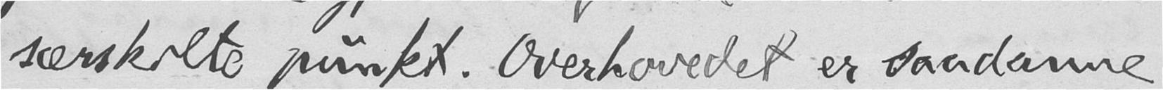særskilte punkt. Overhovedet er saadanne
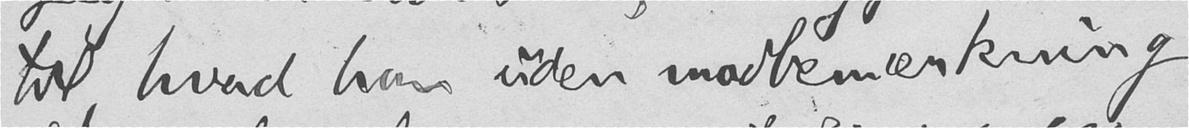til, hvad han uden modbemærkning
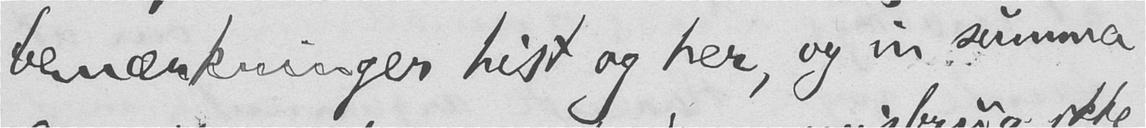bemærkninger hist og her, og in summa
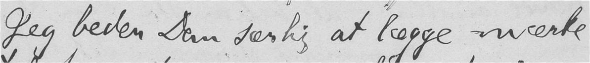Jeg beder Dem særlig at lægge mærke
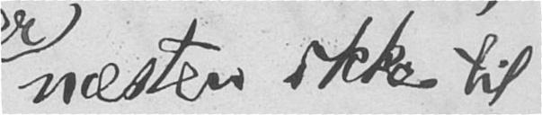næsten ikke til-
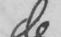de
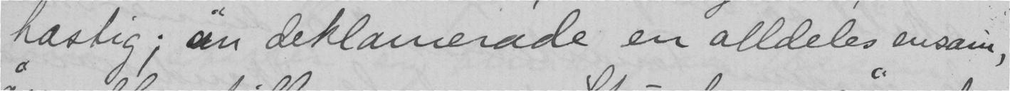hastig; än deklamerade en alldeles ensam,
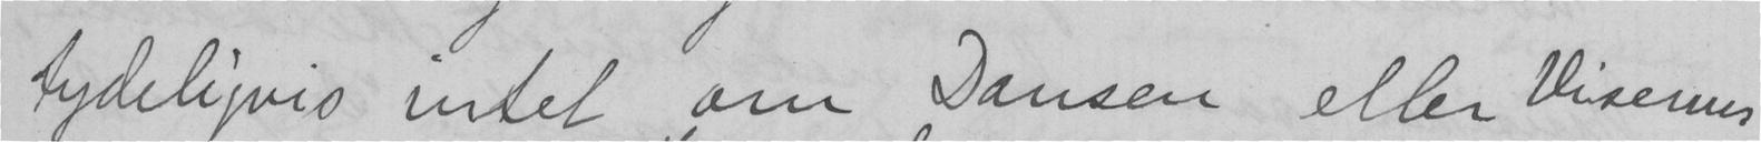tydeligvis intet om Dansen eller Visernes
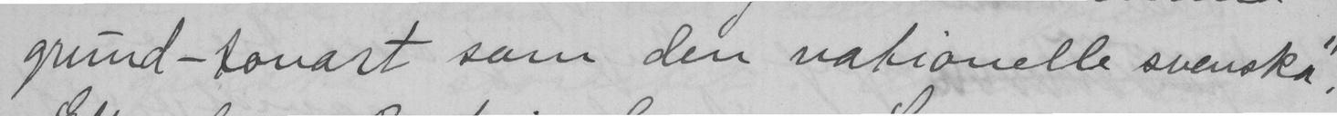grund-tonart som den nationelle svenska".
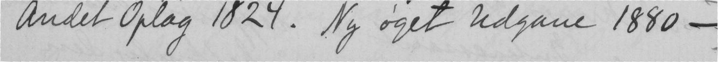Andet Oprag 1824. Ny øget Udgave 1880 -
